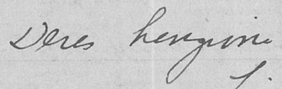Deres hengivne
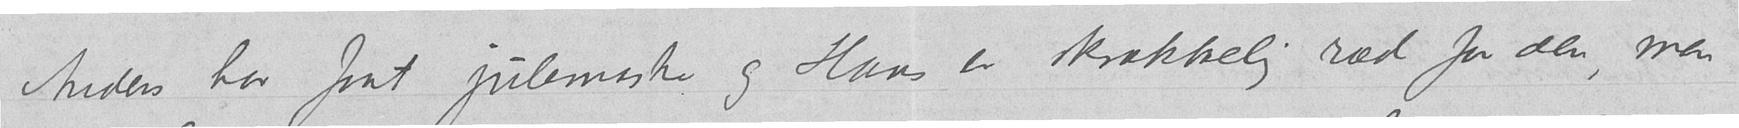Anders har faat julemaske og Hans er skrækkelig ræd for den, men
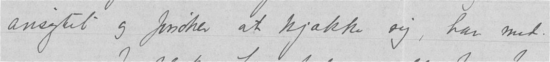ansigtet og forsøker at kjække sig, han med.
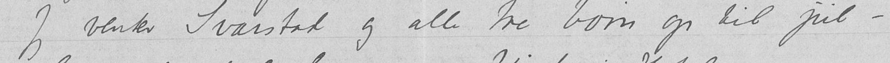Jeg venter Svarstad og alle tre børn op til jul –
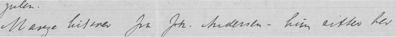Mange hilsener fra frk. Andersen – hun sitter her
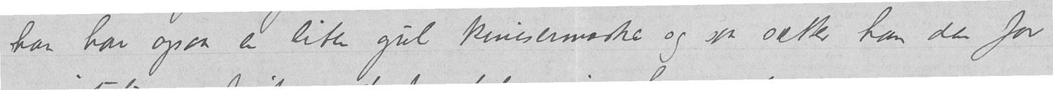han har ogsaa en liten gul kinesermasker og saa sætter han den for
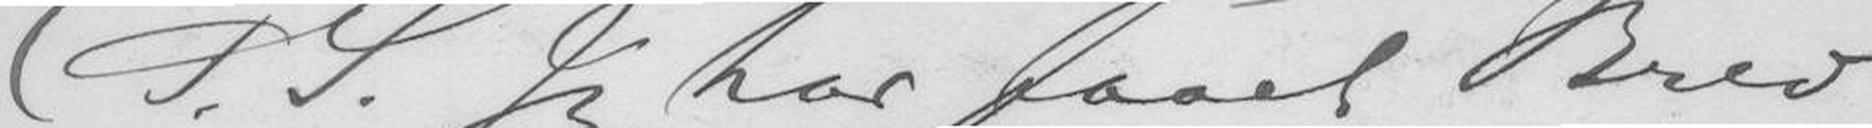(P. S. Jeg har faaet Brev
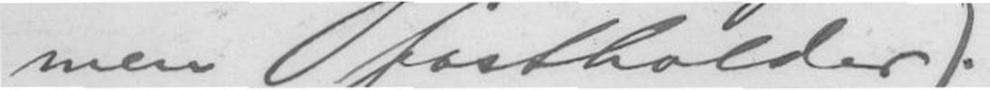men fastholder).
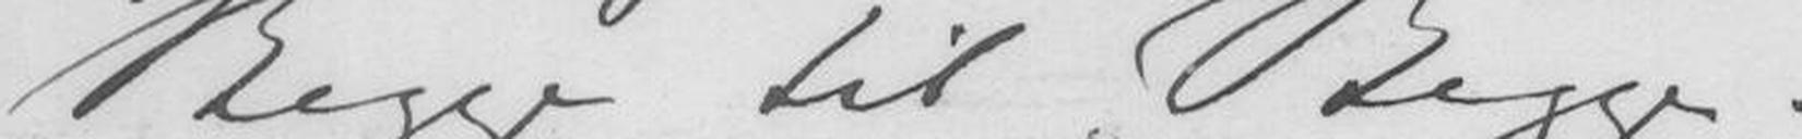Begge til Begge.
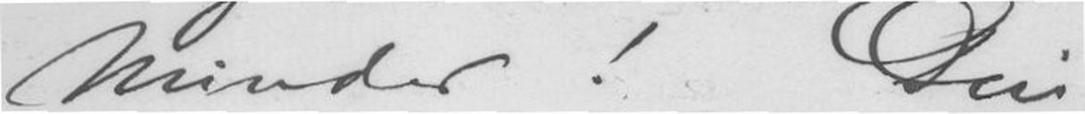Minder! Din
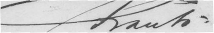Frants.
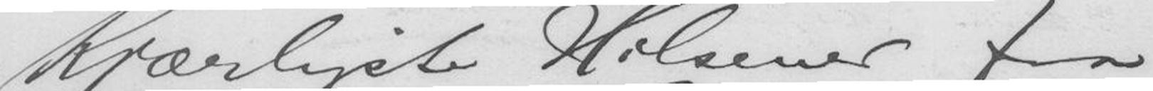Kjærligste Hilsener fra
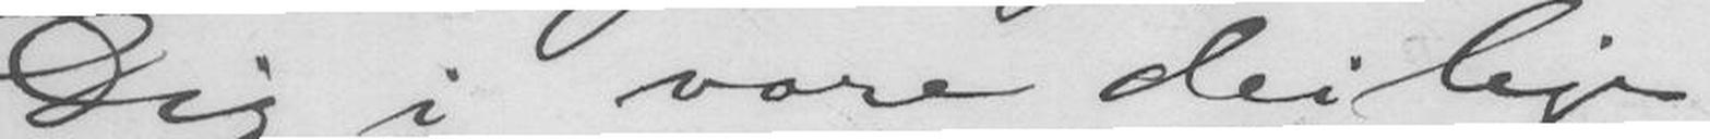Dig i vore deilige
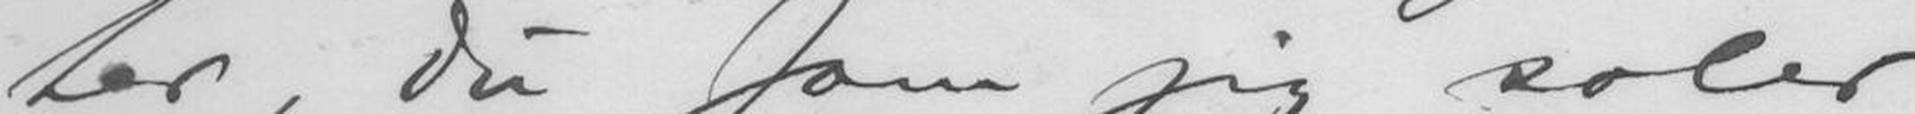ker, du som jeg soler
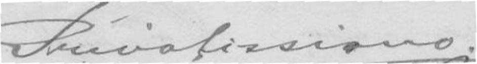Privatissimo.
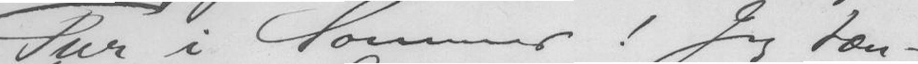Tur i Sommer! Jeg tæn-
